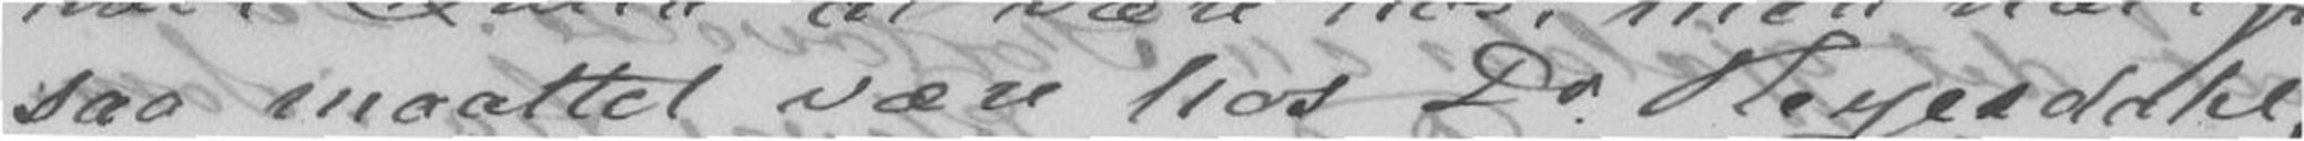saa maattet være hos Dr Heyerdahl,
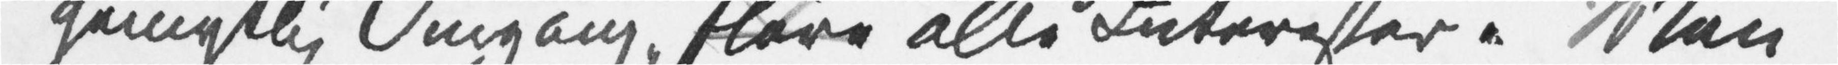gemytlig Omgang, sløve alle Interesser. Man
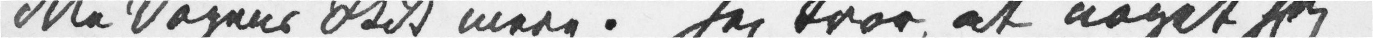ikke Dagens Skik mere. Jeg tror, at noget jeg
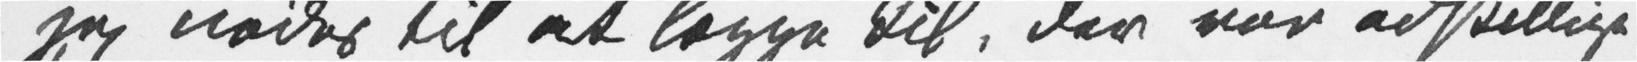jeg nødes til at lægge til, der var adskillige
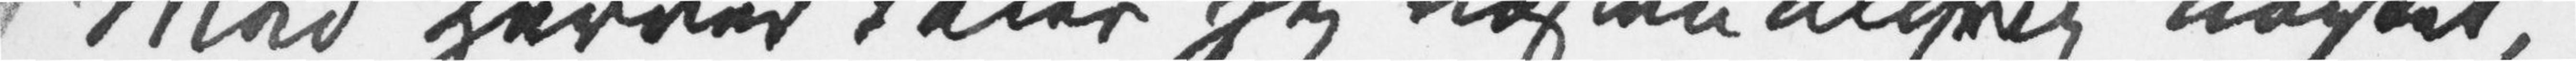Med Herrer taler jeg næsten aldrig uagtet,
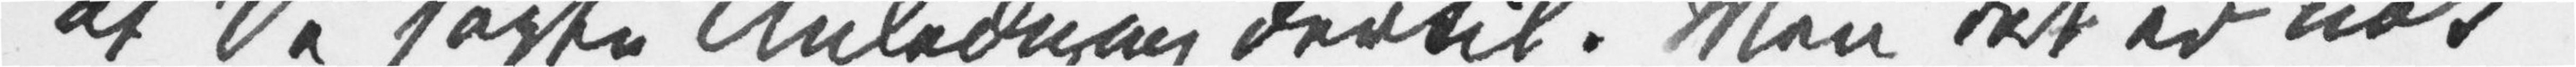at De søgte Anledning dertil. Men det er nok
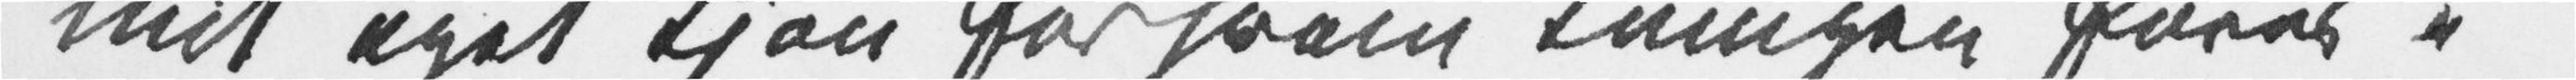mit eget Kjøn for hvem Kampen føres.
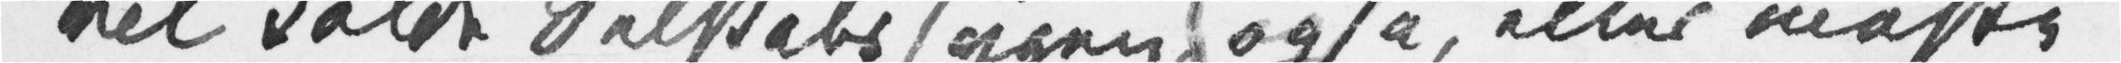vil kalde Selskabssygen, også, eller måske
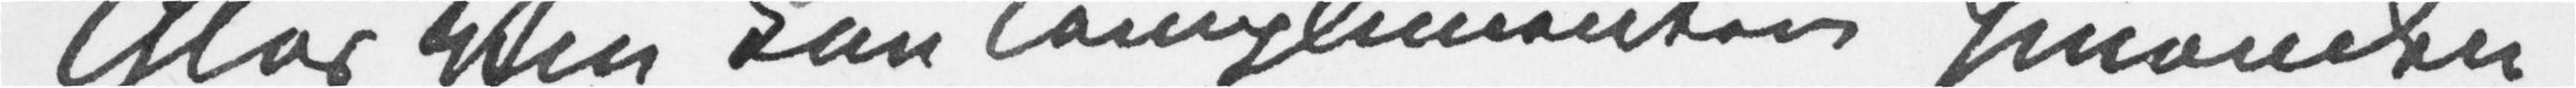Glas Vin kan komplimentere hinanden
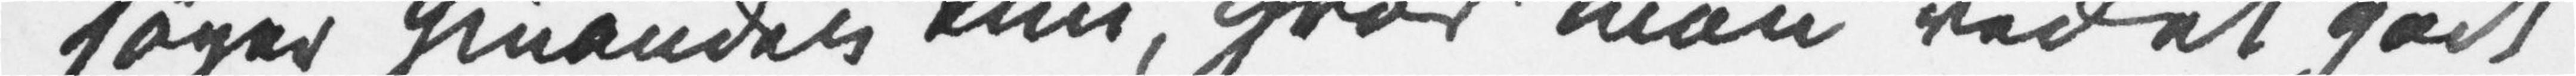søger hinanden kun, hvor man ved et godt
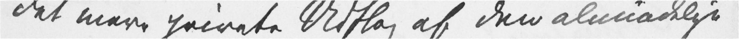det mere private Udslag af den almindelige
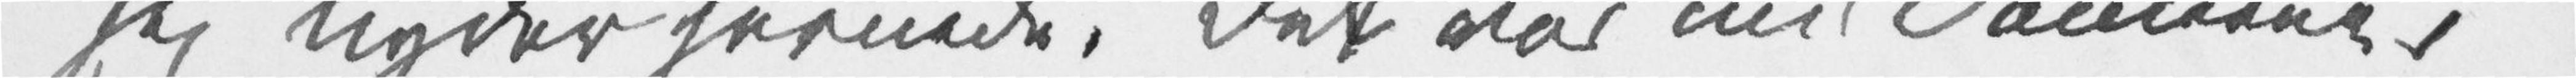jeg nyder hernede. Det var nu Damerne,
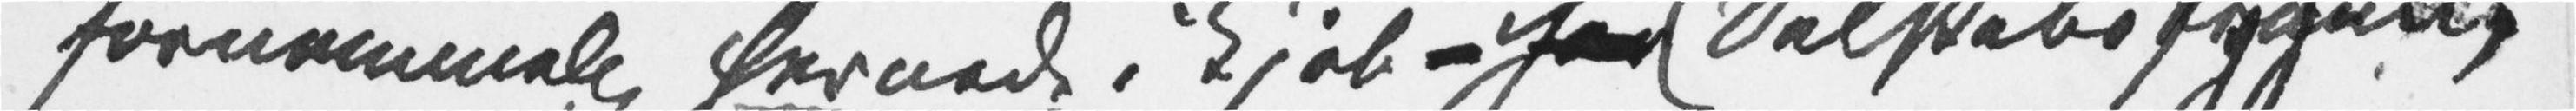fornemmelig hernede i Kjøb – her (Selskabssygen,
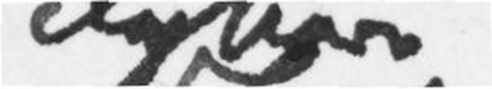dybere
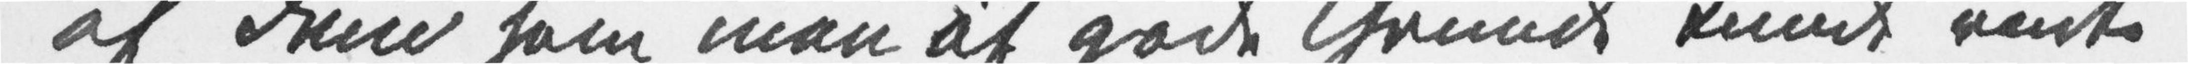af dem som man af gode Grunde kunde vente
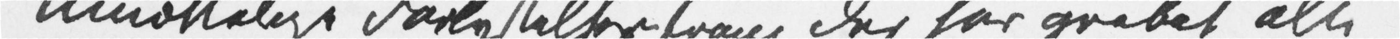umættelige Forlystelsestrang der har grebet alle
